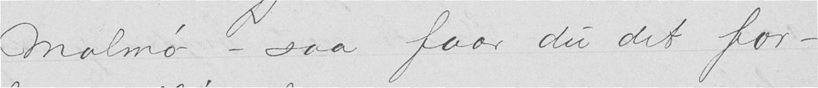Malmø - saa faar du det for-
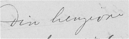Din hengivne
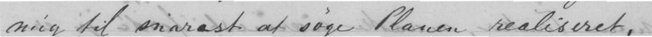mig til snarest at søge Planen realiseret,
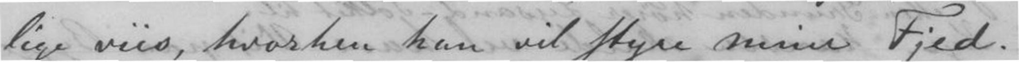lige viis hvorhen han vil styre mine Fjed.
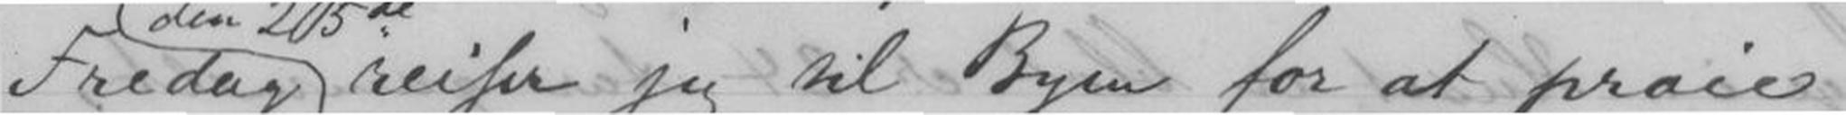Fredag reiser jeg til Byen for at praie
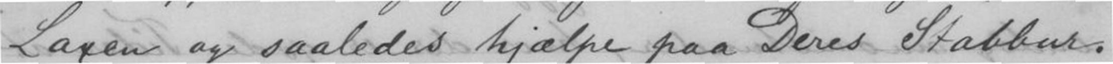Laxen og saaledes hjælpe paa Deres Stabbur.
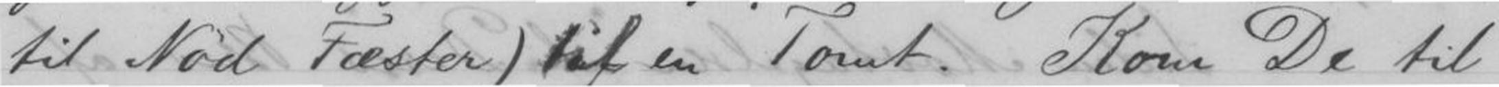til Nød Fæster) til en Tomt. Kom De til
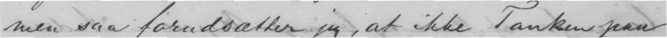men saa forudsætter jeg, at ikke Tanken paa
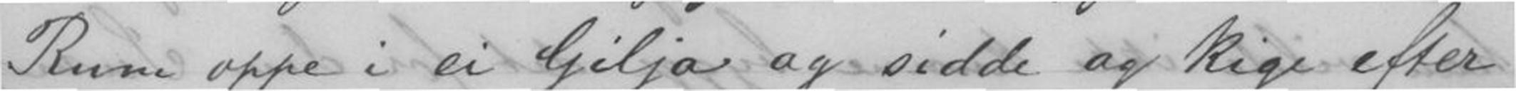Rum oppe i ei Gilja og sidde og kige Efter
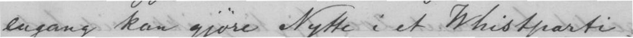engang kan gjøre Nytte i et Whistparti;
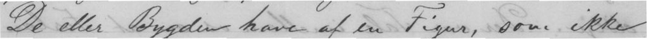De eller Bygden have af en Figur, som ikke
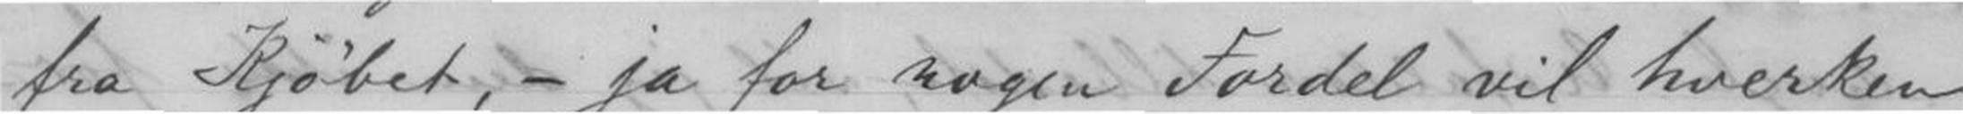fra Kjøbet, - ja for nogen Fordel vil hverken
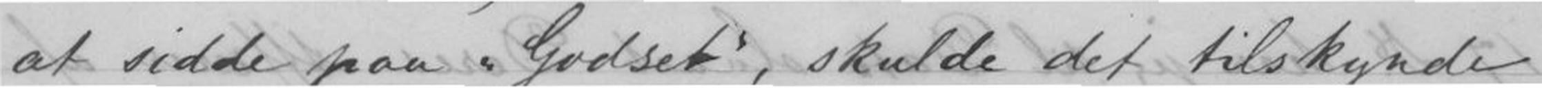at sidde paa Godset, skulde det tilskynde
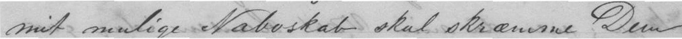mit mulige Naboskab skal skræmme Dem
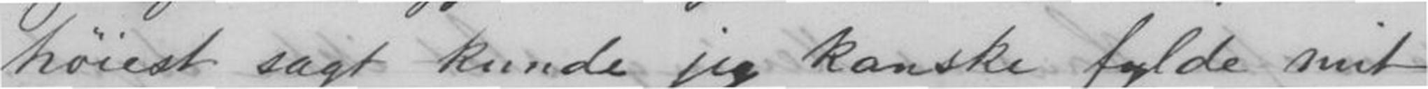høiest sagt kunde jeg kanske fylde mit
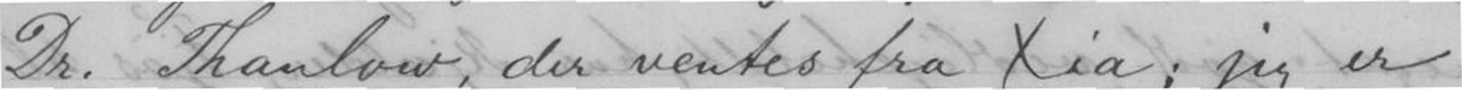Dr. Thaulow, der ventes fra Xia; jeg er
